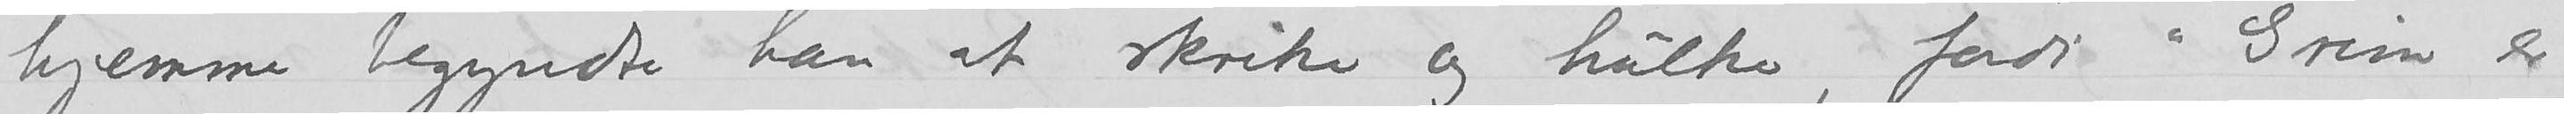hjemme begyndte han at skrike og hulke, fordi «Grim er
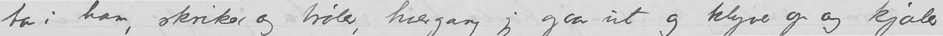tar i ham, skriker og brøler, hvergang jeg gaar ut og klyver op og
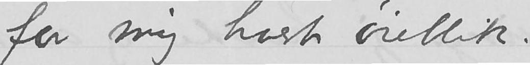kjæler for mig hvert øieblik.
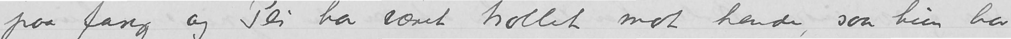paa fang og Pei har været trollet mot hende, saa hun har
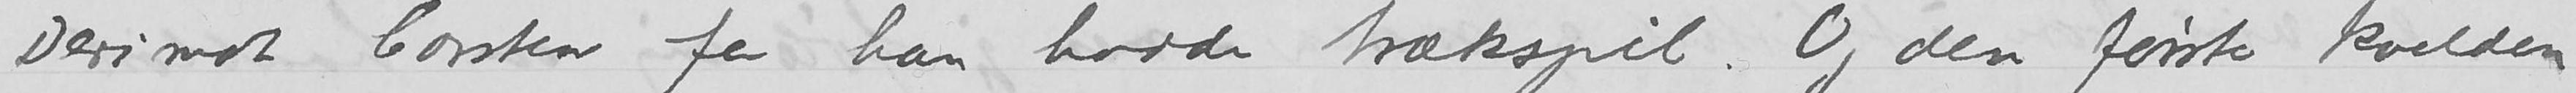Derimot Carsten for han hadde trækspil. Og den første kvelden
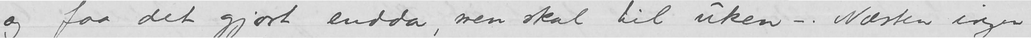ned og faa det gjort endda, men skal til uken -. Næsten ingen
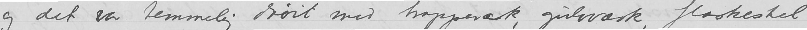og det var temmelig drøit med trappevask, gulvvask, flaskestel,
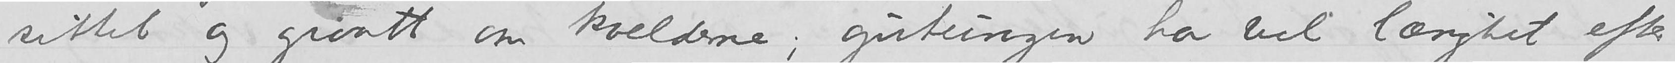sittet og graatt om kvelderne; gutungen har vel længtet efter
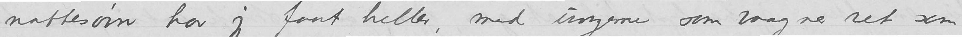nattesøvn har jeg faat heller, med ungerne som vaagner ret som
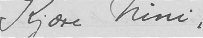Kjære Nini,
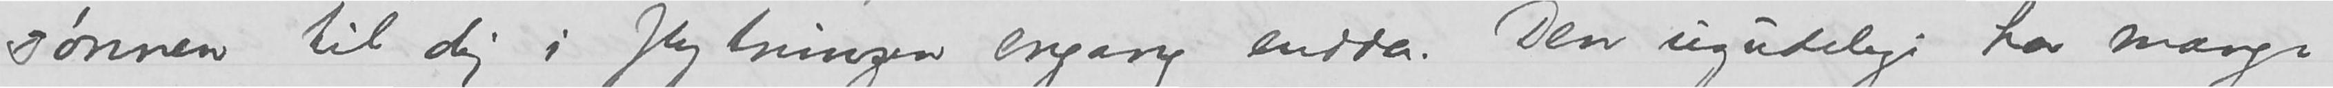sønnen til dig i flytningen engang endda. Den ugudelige har mange
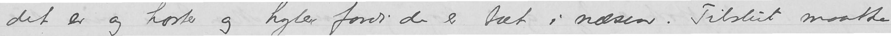det er og hoster og hyler fordi de er tæt i næsen. Tilslut maatte
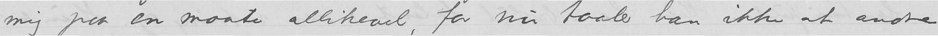mig paa en maate allikevel, for nu taaler han ikke at andre
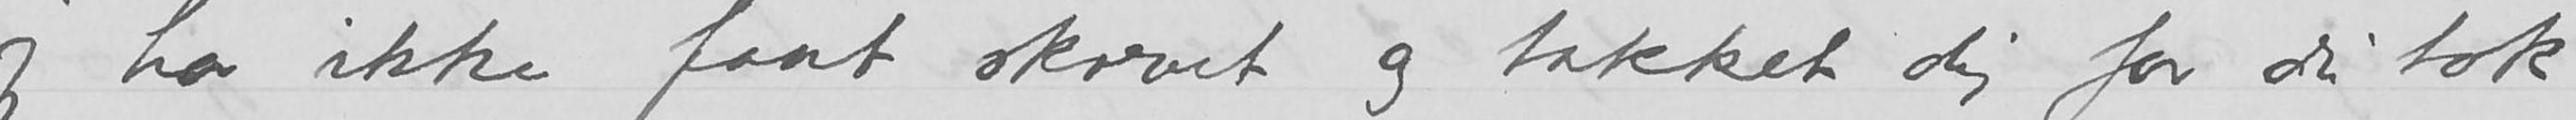jeg har ikke faat skrevet og takket dig for du tok
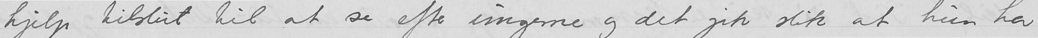hjelp tilslut til at se efter ungerne og det gik slik at hun har
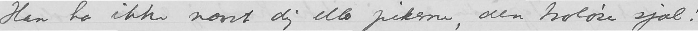Han har ikke nævnt dig eller pikerne, den troløse sjæl!
